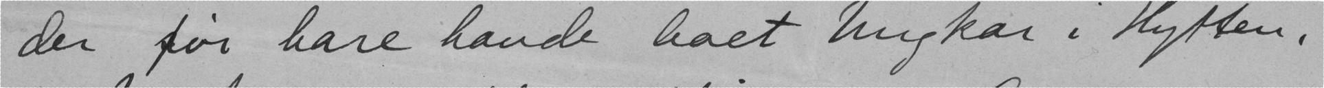der før bare havde boet Ungkar i Hytten,
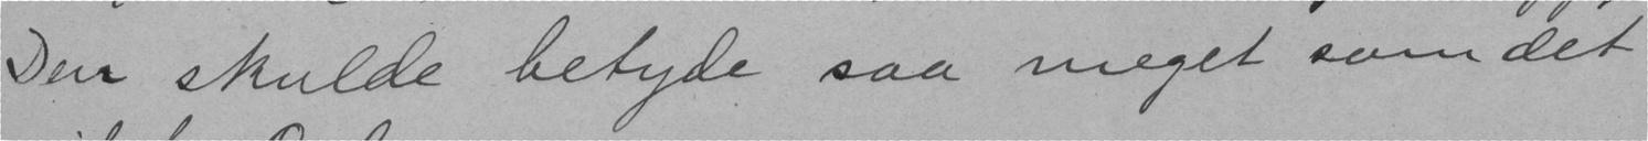Den skulde betyde saa meget som det
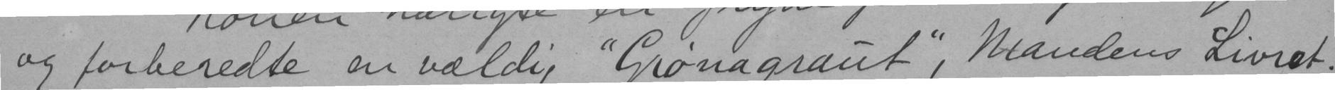og forberedte en vældig "Grønagraut", Mandens Livret.
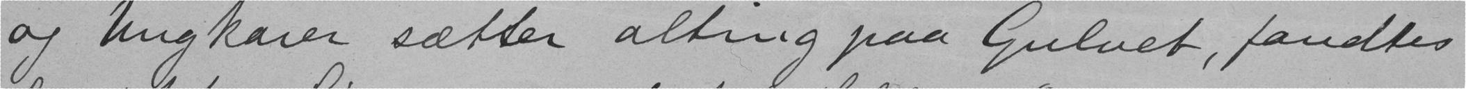og Ungkarer sætter alting paa Gulvet, fandtes
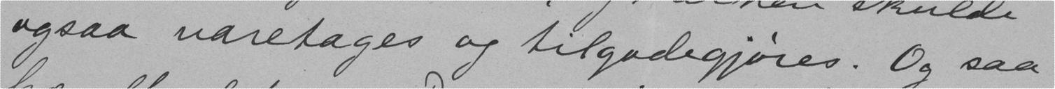ogsaa varetages og tilgodegjøres. Og saa
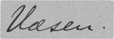Væsen.
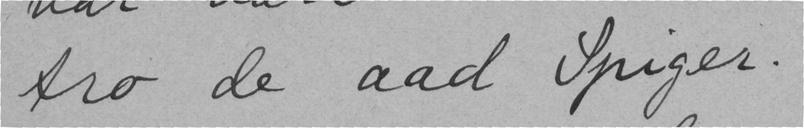tro de aad Spiger.
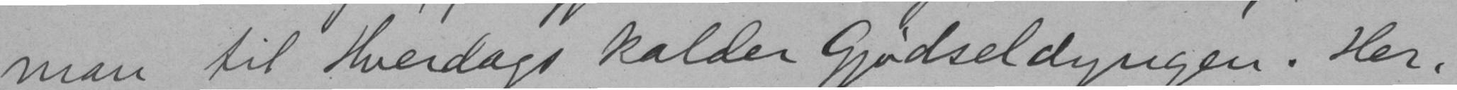man til Hverdags kalder Gjødseldyngen. Her,
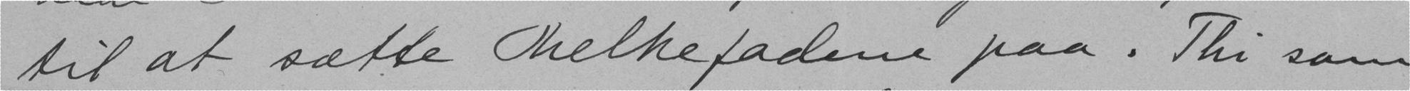til at sætte Melkefadene paa. Thi som
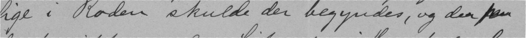lige i Roden skulde der begyndes, og der paa
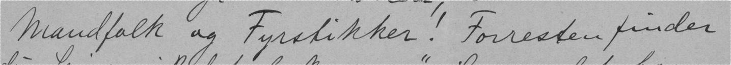Mandfolk og Fyrstikker! Forresten finder
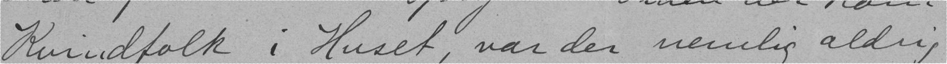Kvindfolk i Huset, var der nemlig aldrig
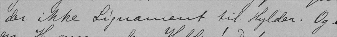der ikke Lignament til Hylder. Og
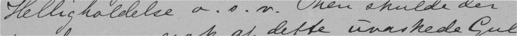Helligholdelse o. s. v. Men skulde der
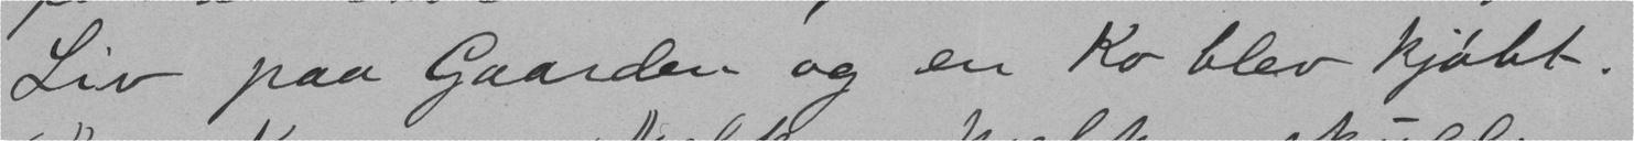Liv paa Gaarden og en Ko blev kjøbt.
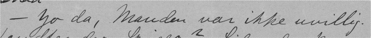- Jo da, Manden var ikke uvillig.
